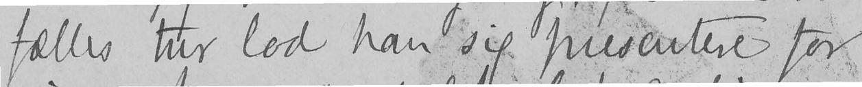fælles tur lod han sig presentere for
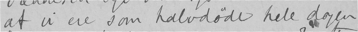at vi ere som halvdøde hele dagen
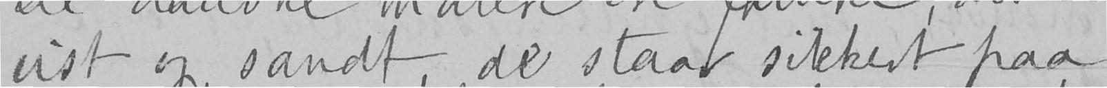vist og sandt, de staar sikkert paa
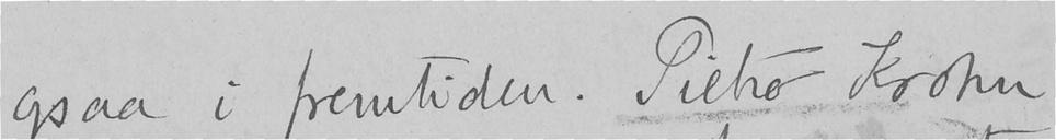ogsaa i fremtiden. Pietro Krohn
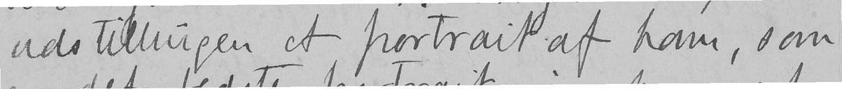udstillingen et portrait af ham, som
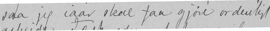saa jeg iaar skal faa gjøre ordenligt
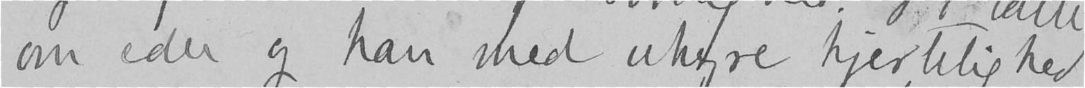om eder og han med uhyre hjertelighed
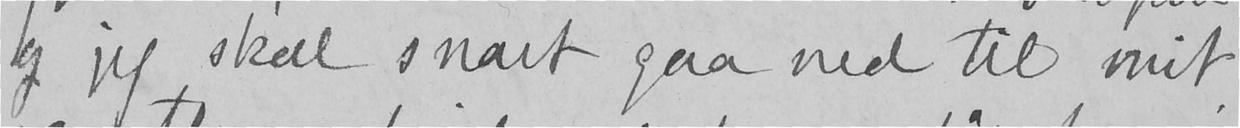og jeg skal snart gaa ned til mit
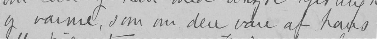og varme, som om dere vare af hans
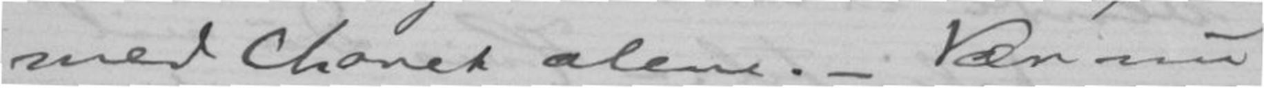med Choret alene.- Vær nu
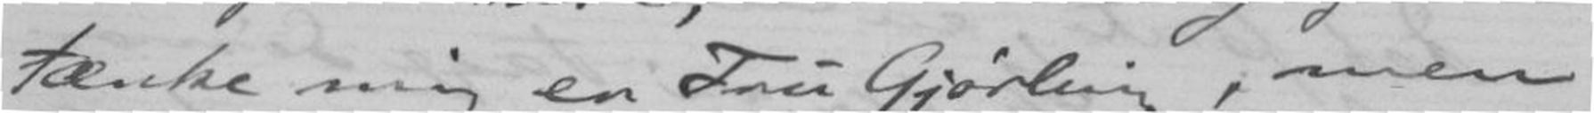tænke mig er Fru Gjørling, men
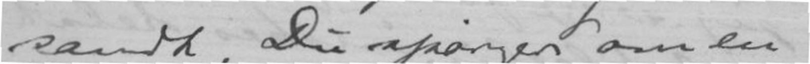sandt, Du spørger om en
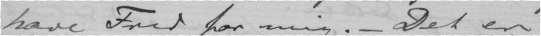have Fred for mig.- Det er
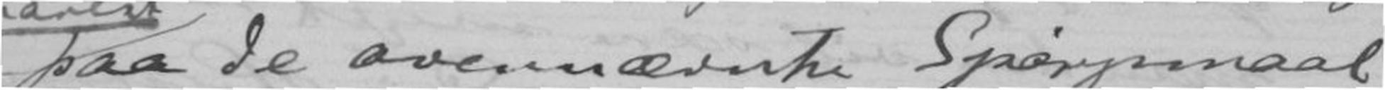paa de ovennævnte Spørgsmaal
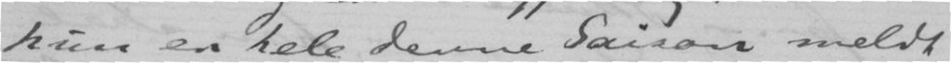hun er hele denne Saison meldt
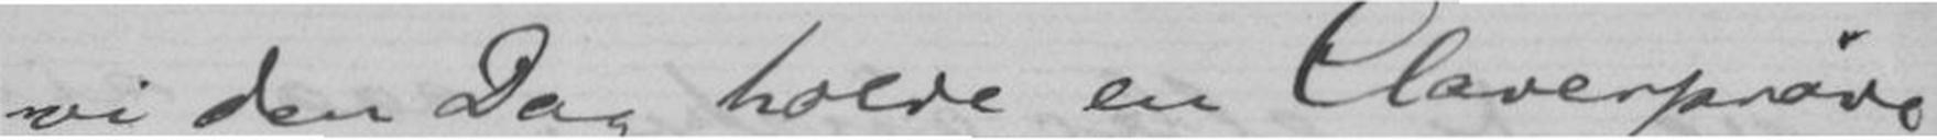vi den Dag holde en Claverprøve
